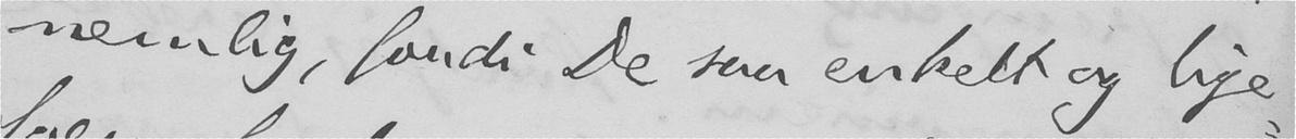nemlig, fordi De saa enkelt og lige-
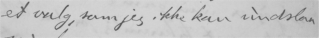et valg, som jeg ikke kan undslaa
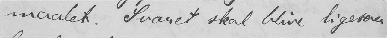maalet. Svaret skal blive ligesaa
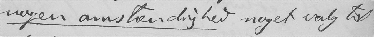nogen omstændighed noget valg til
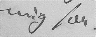mig for.
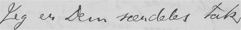Jeg er Dem særdeles tak-
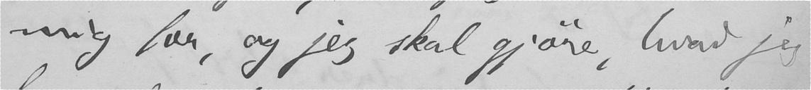mig for, og jeg skal gjøre, hvad jeg
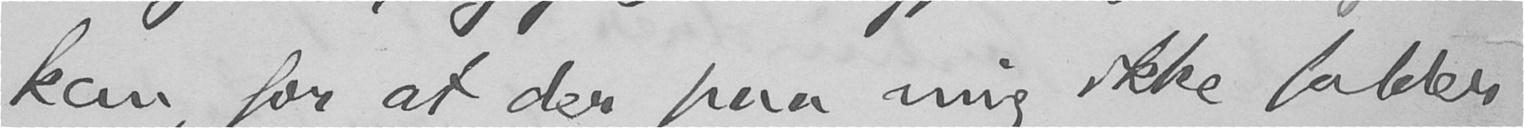kan, for at der paa mig ikke falder
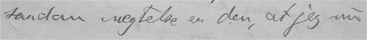saadan nægtelse er den, at jeg nu
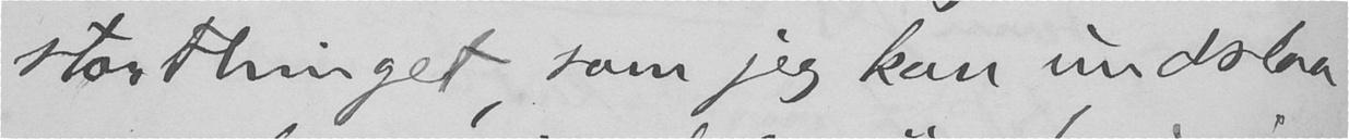storthinget, som jeg kan undslaa
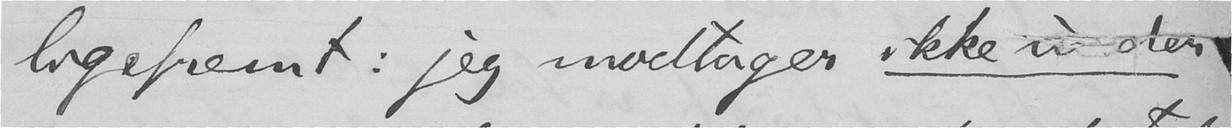ligefremt: jeg modtager ikke under
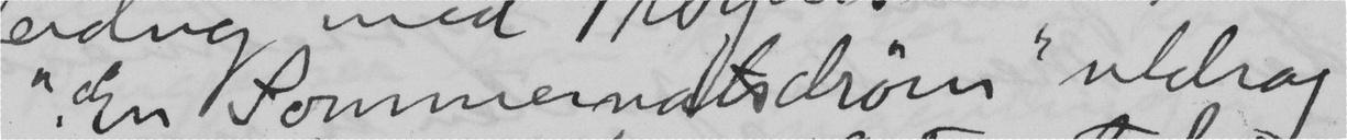"En Sommernatsdröm" utdrag
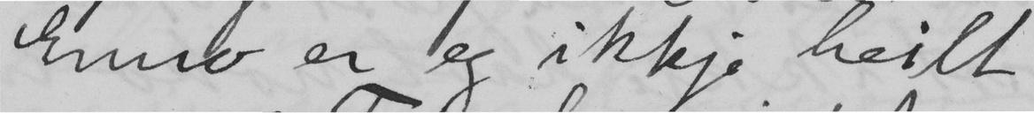Enno er jeg ikkje heilt
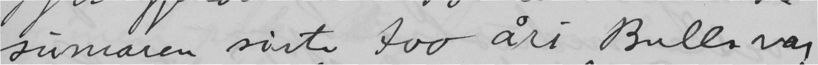sumaren siste tvo åri Bulls var
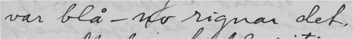var blå – no rignar det.
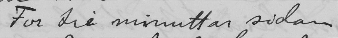For tri minuttar sidan
